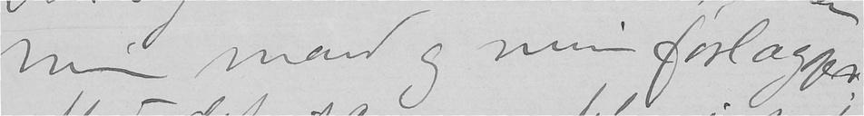min mand og min forlægger.
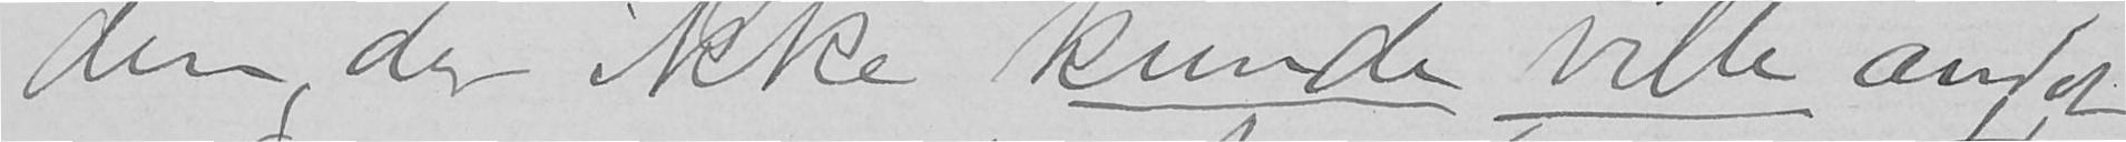den, der ikke kunde ville andet
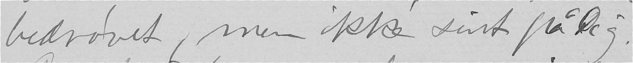bedrøvet, men ikke sint på Dig.
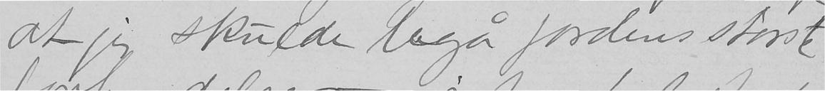at jeg skulde begå jordens største
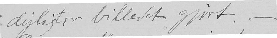dejligt er billedet gjort. -
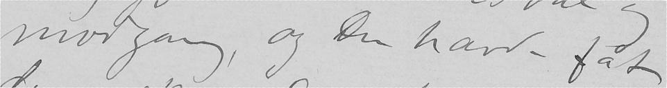modgang, og Du havde fåt
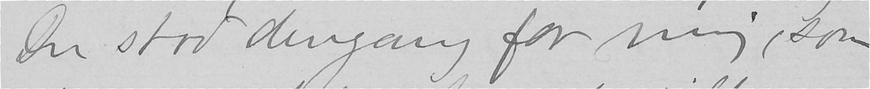Du stod dengang for mig, som
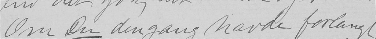Om Du dengang havde forlangt
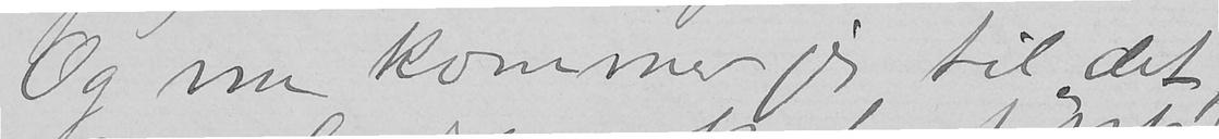Og nu kommer jeg til det
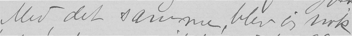Med det samme, blev jeg nok
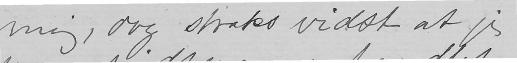mig, dog straks vidst at jeg
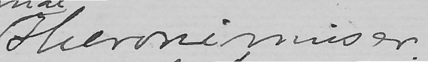Hieronimus er .
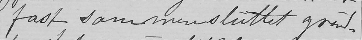fast sammensluttet grund-
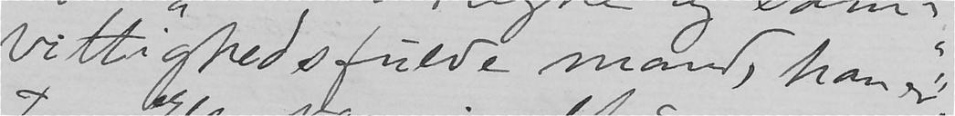vittighedsfulde mand, han er".
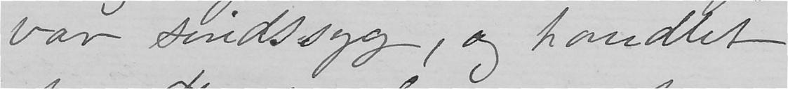var sindssyg, og handlet
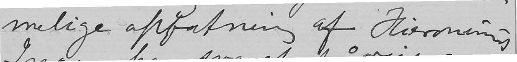melige opfatning af Hieronimus.
